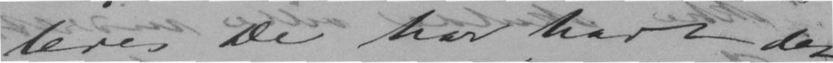ledes De har havt det
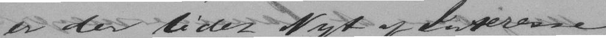er der lidet Nyt af Interesse
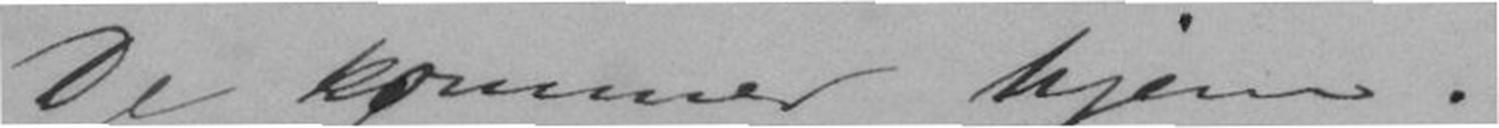De kommer hjem.
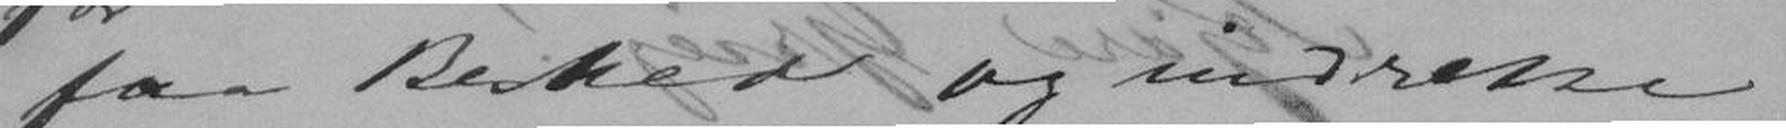faa Besked og indrette
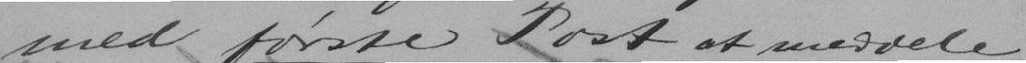med første Post at meddele
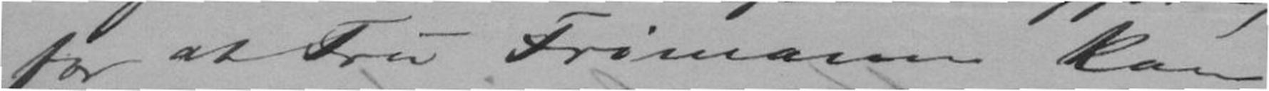for at Fru Frimann kan
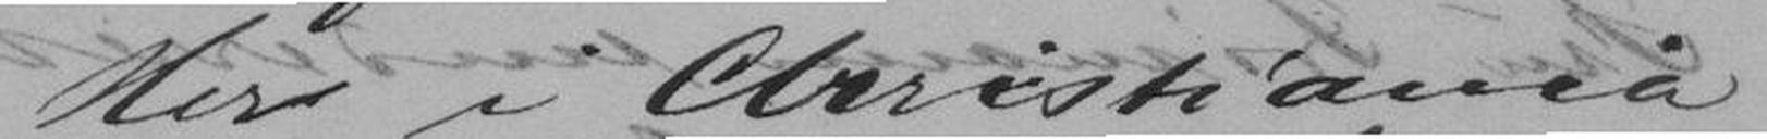Her i Christiania
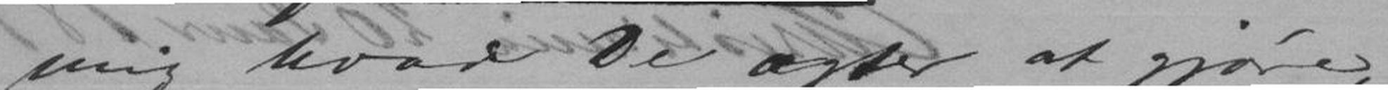mig hvad De agter at gjøre,
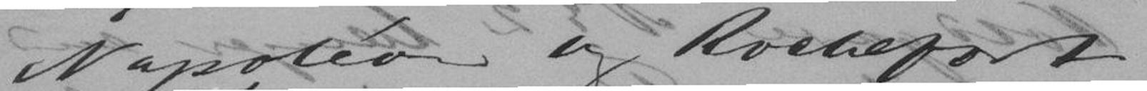Napolèon og Rochefort.
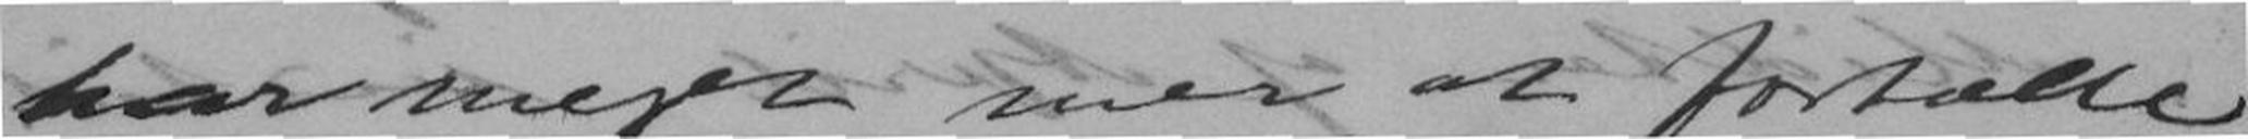har meget mer at fortælle
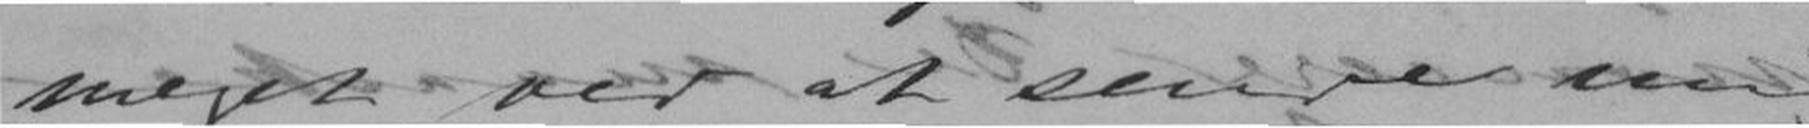meget ved at sende mig
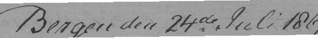Bergen den 24de Juli 1867.
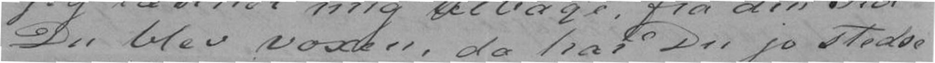Du blev voxen, da har Du jo stedse
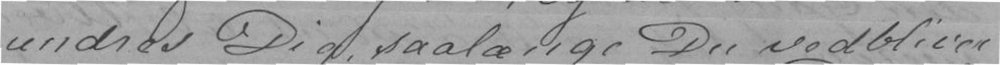undres Dig saalænge Du vedbliver
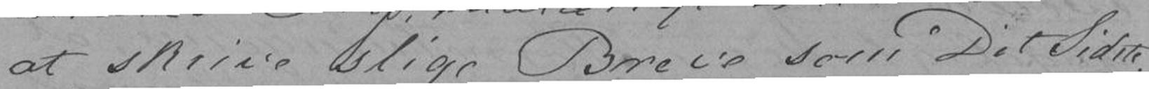at skrive slige Breve som Dit Sidste.
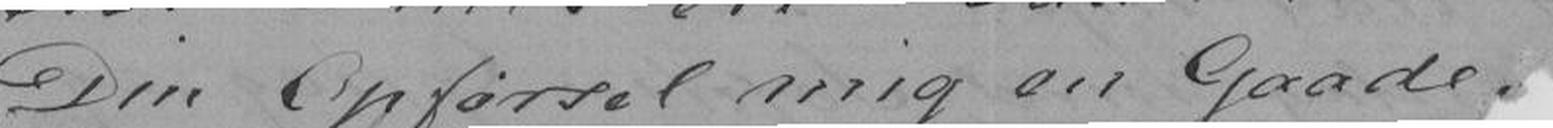Din Opførsel mig en Gaade.
